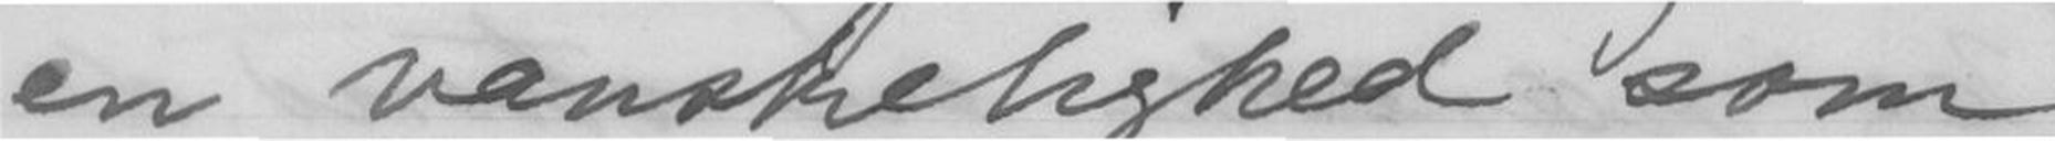en vanskelighed som
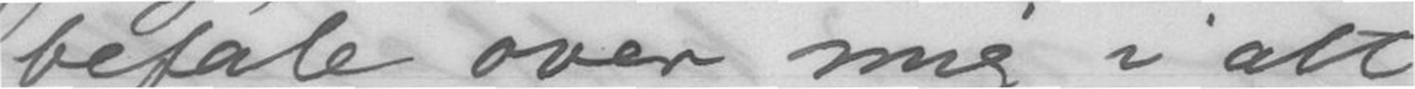befale over mig i alt
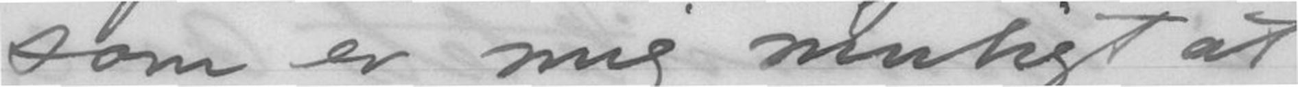som er mig muligt at
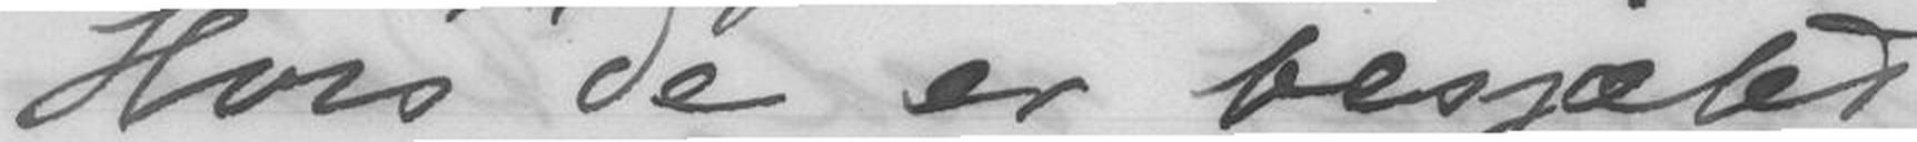Hvis De er besjælet
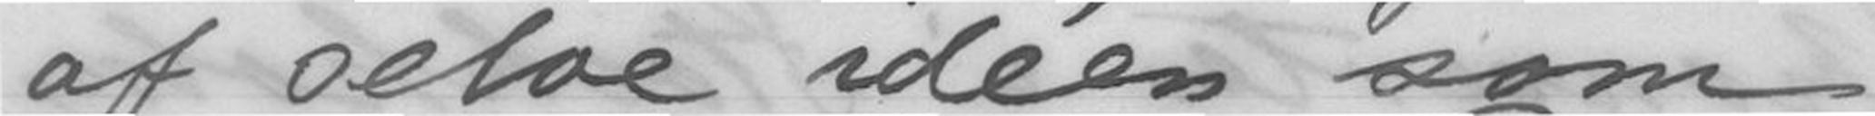af selve ideen som
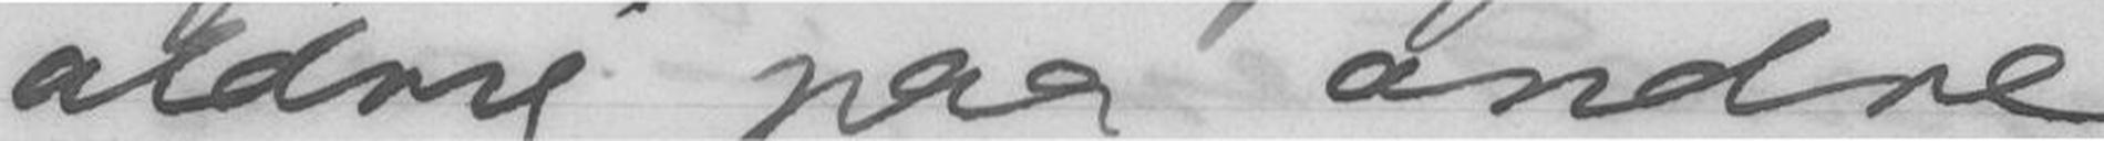aldrig paa andre
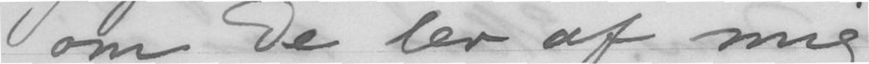om De ler af mig
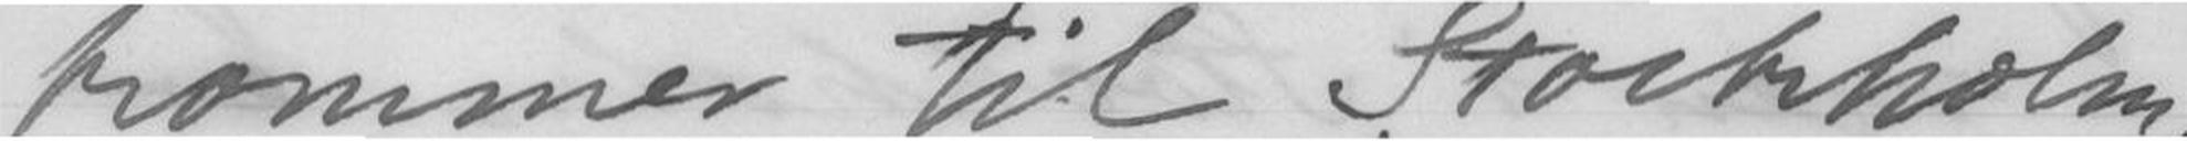kommer til Stockholm,
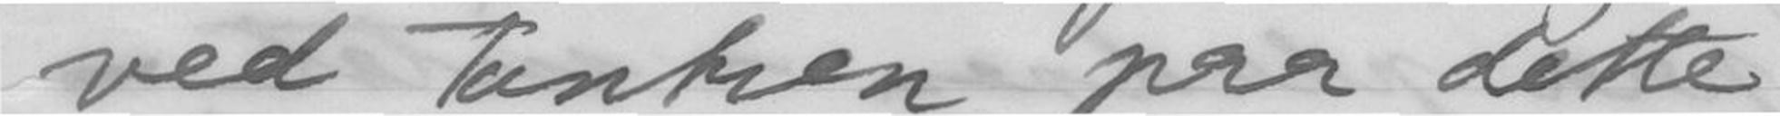ved tanken paa dette
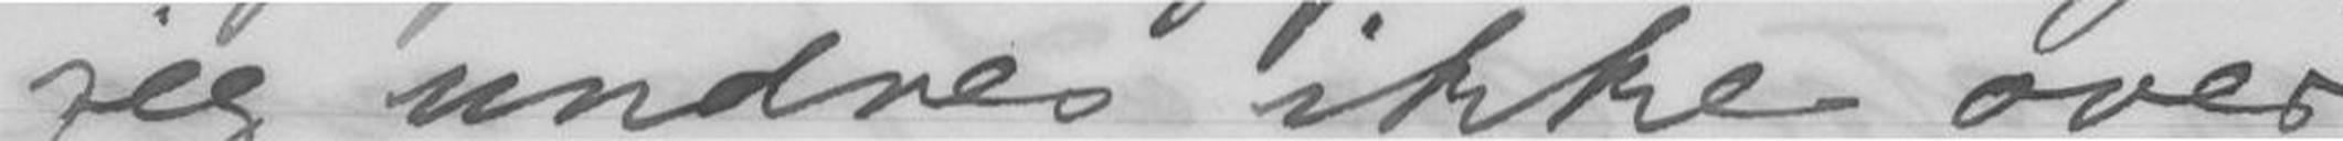jeg undres ikke over
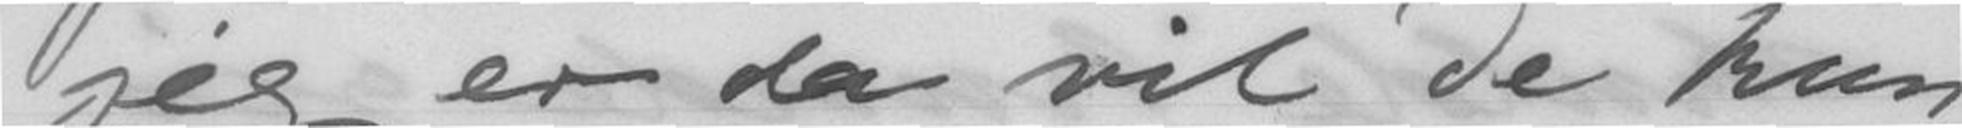jeg er da vil De kun
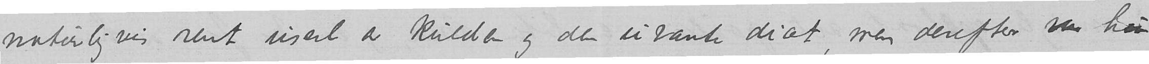naturligvis rent usel av kulden og den uvante diæt, men derefter var hun
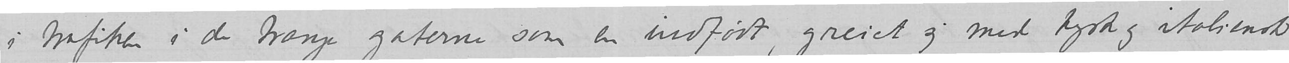i trafiken i de trange gaterne som en indfødt, greiet sig med tysk og italiensk
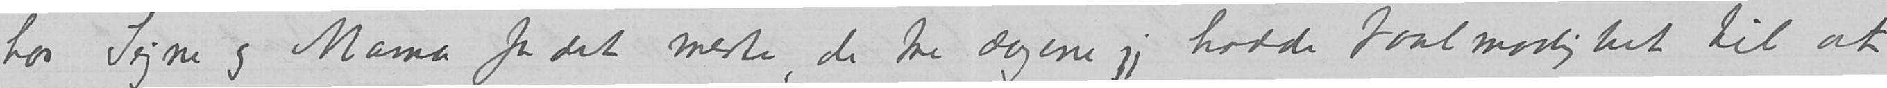hos Signe og Mama for det meste, de tre dagene jeg hadde taalmodighet til at
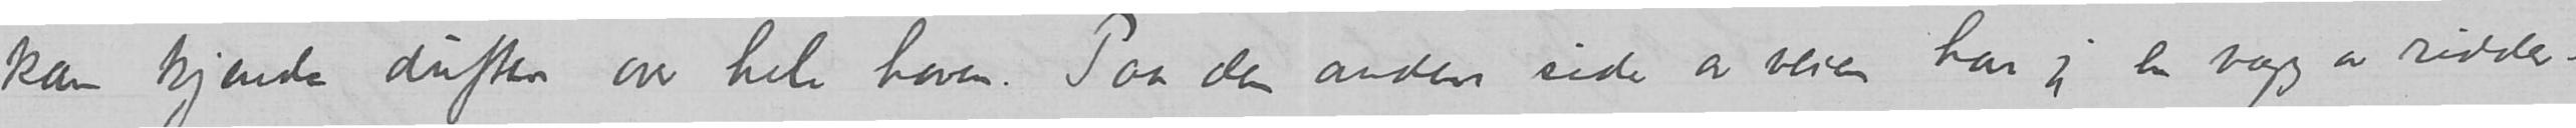kan kjende duften av hele haven. Paa den anden side av veien har jeg en væg av ridder
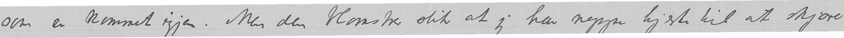Odier som er kommet igjen. Men den blomstrer slik at jeg har neppe hjerte til at skjære
